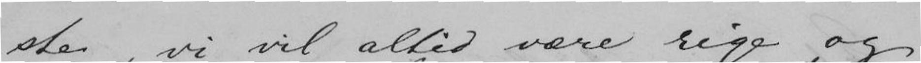ste, vi vil altid være rige og
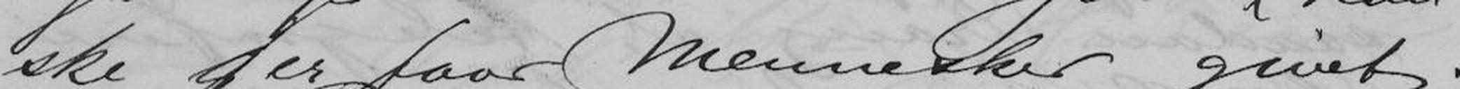ske f er faa Mennesker givet.
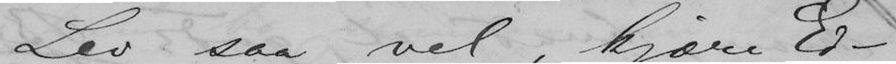Lev saa vel, kjære Ed-
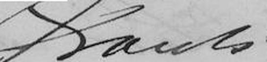Frants.
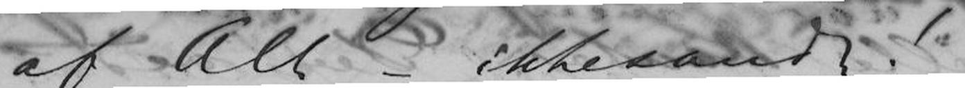af Alt - ikkesandt!
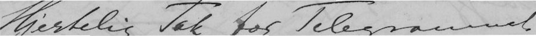Hjertelig Tak for Telegrammet
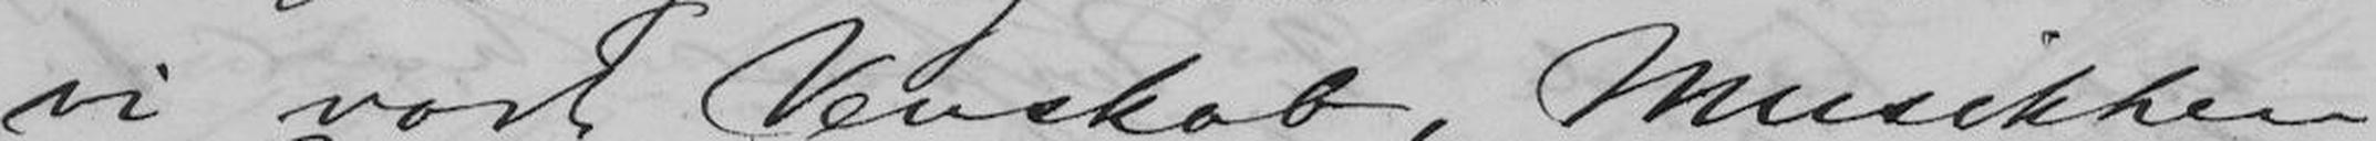vi vort Venskab, Musikken
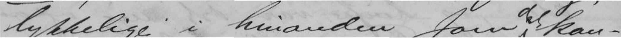lykkelige i hinanden som kan-
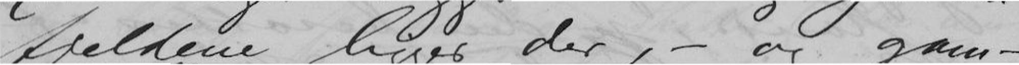fjeldene ligger der, - og gam-
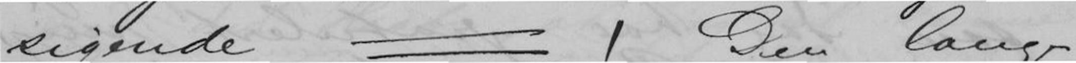sigende Noter: g! Den lange
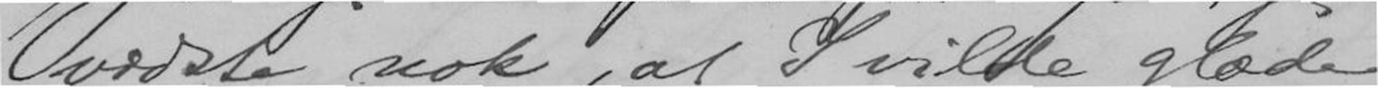vidste nok, at I vilde glæde
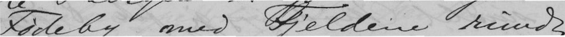Fødeby med Fjeldene rundt
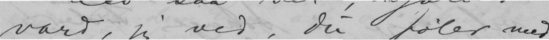vard, jeg ved, Du føler med
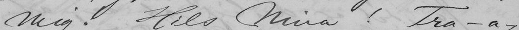mig. Hils Nina! Tra-a-
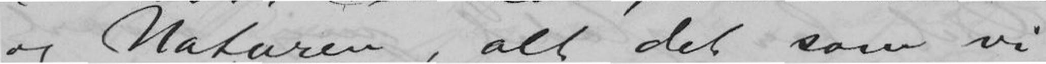og Naturen, alt det som vi
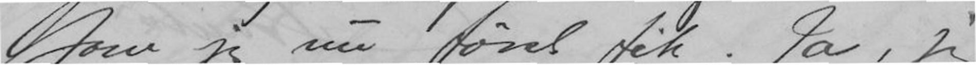som jeg nu først fik. Ja, jeg
